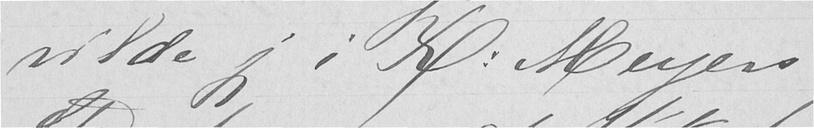vilde jeg i R. Meyers
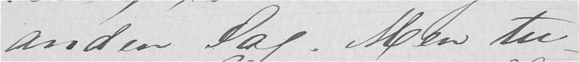anden Sag. Men tu-
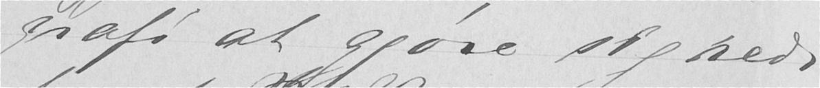grafi at gjøre sig rede
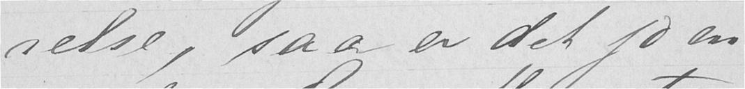relse, saa er det jo en
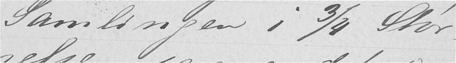Samlingen i 3/4 Stør-
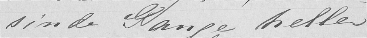sinde Gange heller
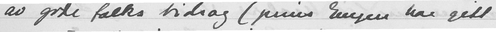av gode folks bidrag (prins Eugen har gitt
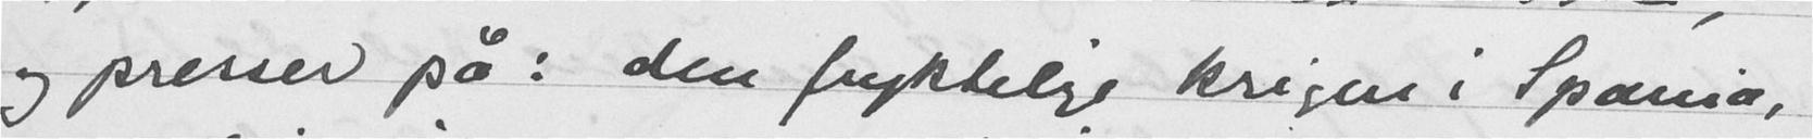og presser på: den fryktelige krigen i Spania,
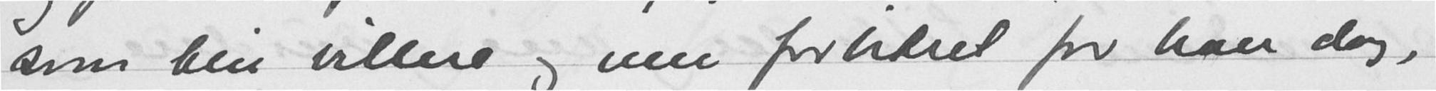som blir villere og mer forbitret for hver dag,
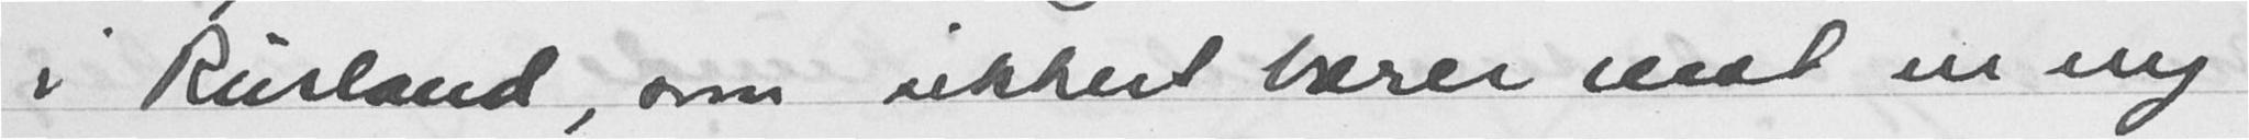i Rusland, som sikkert bærer mot en ny
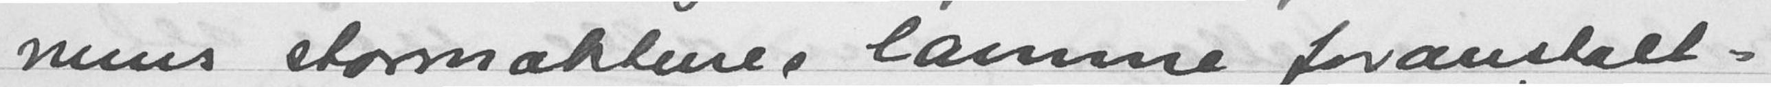mens stormaktenes lamme foranstalt-
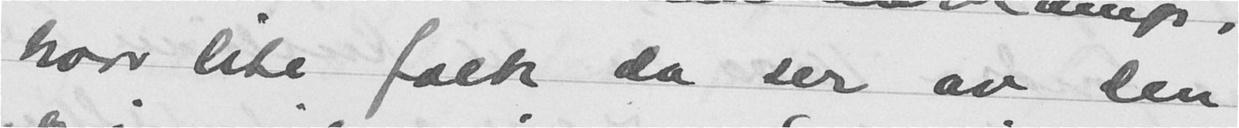hvor lite folk da ser av den
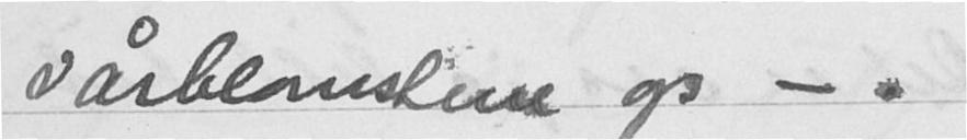vårblomstene op -.
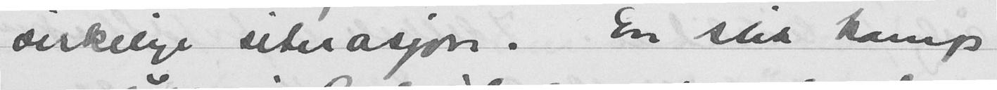virkelige situasjon. En slik kamp
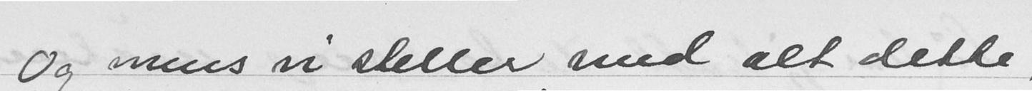Og mens vi stiller med alt dette,
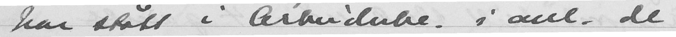har stått i Arbeidbe i anl. de
